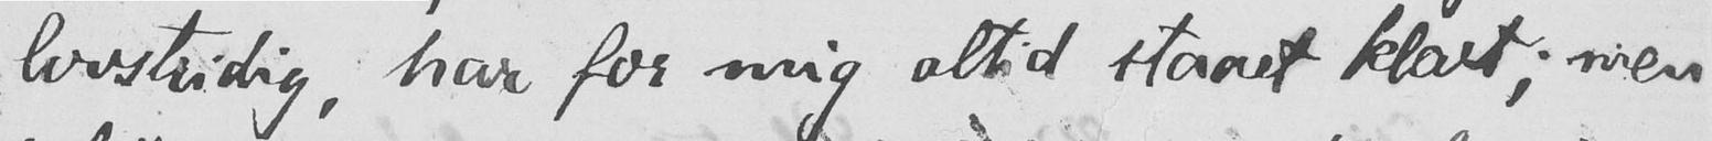lovstridig, har for mig altid staaet klart; men
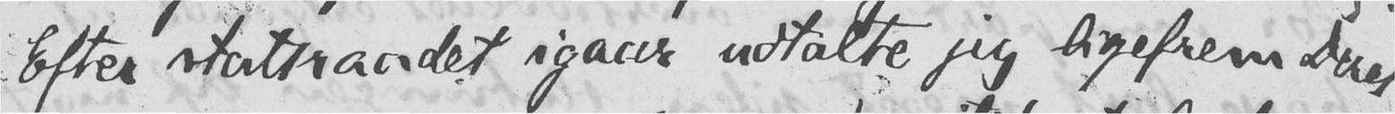Efter statsraadet igaar udtalte jeg ligefrem Deres
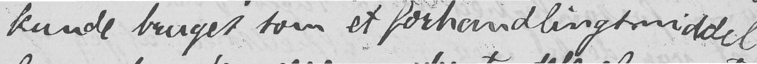kunde bruges som et forhandlingsmiddel
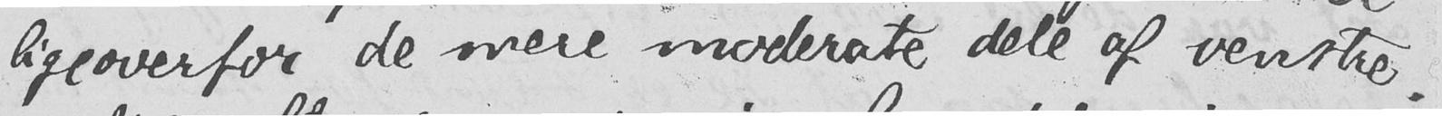ligeoverfor de mere moderate dele af venstre.
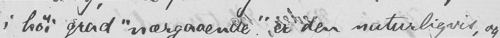i høi grad "nærgaaende" er den naturligvis, og
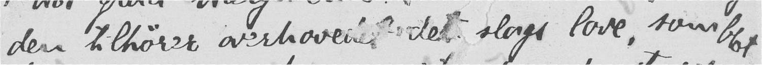den tilhører overhovedet det slags love, som blot
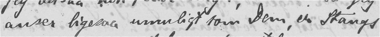anser ligesaa umuligt som Dem, er Stangs
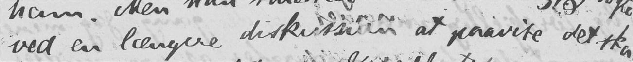ved en længere diskussion at paavise det ska-
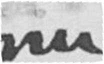nu
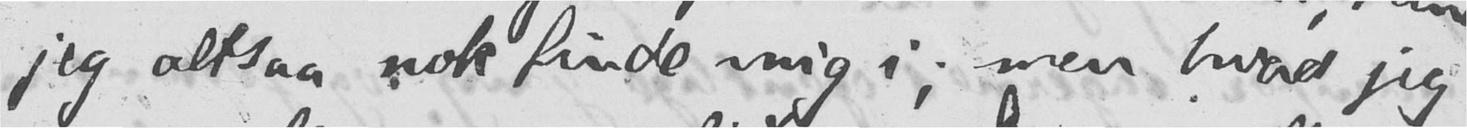jeg altsaa nok finde mig i; men hvad jeg
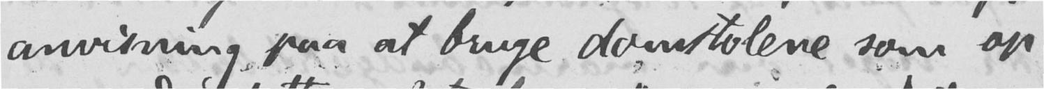anvisning paa at bruge domstolene som op-
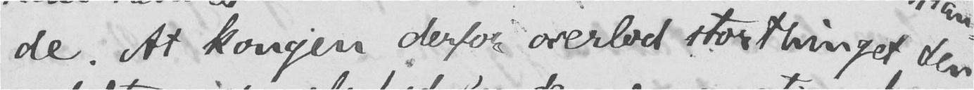de. At kongen derfor overlod storthinget den
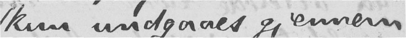kun undgaaes gjennem
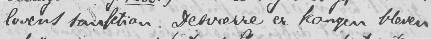lovens sanktion. Desværre er kongen bleven
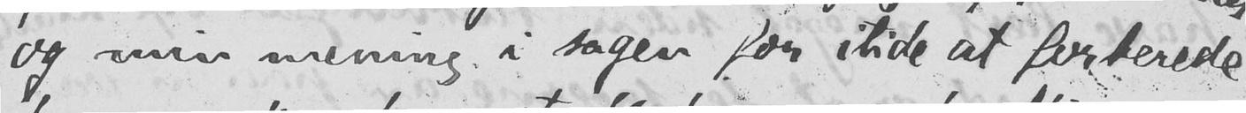og min mening i sagen for itide at forberede
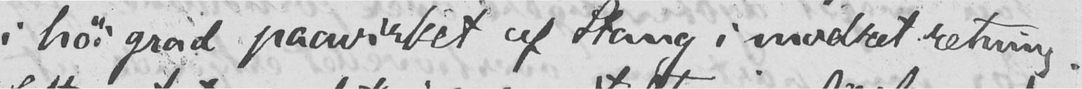i høi grad paavirket af Stang i modsat retning.
